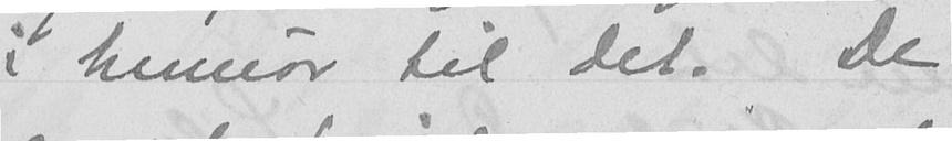i humør til det. De
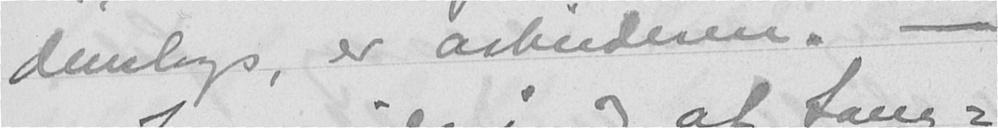denslags, er arbeideren. -
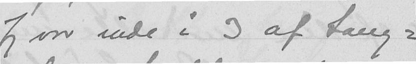Jeg var inde i 3 af Saug-
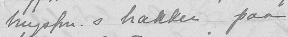brugsforn.s brakker paa
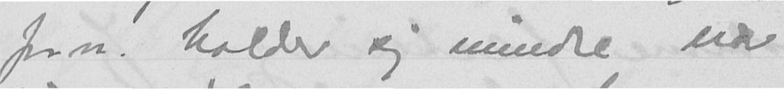forn. holder sig mindre nær
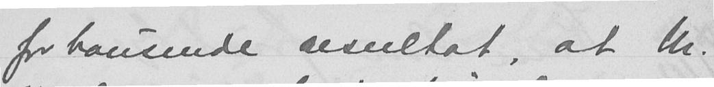forbausende resultat, at M.
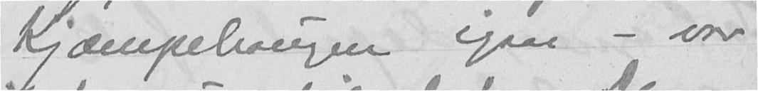Kjæmpehaugen igaar - var
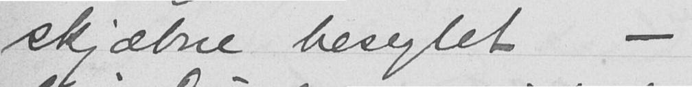skjæbne beseglet -
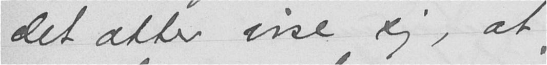det atter vise sig, at
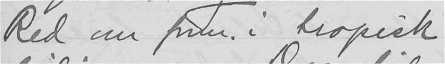Red om form. i tropisk
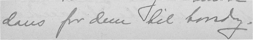dans for dem til torsdag.
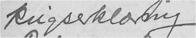krigserklæring.
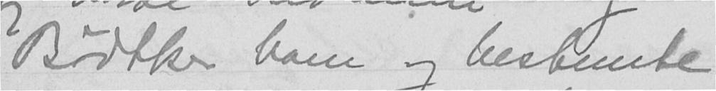Bødtker barn og bestemte
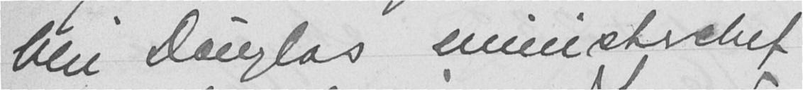blir Douglas ministerchef
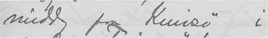middag paa Knivsø i
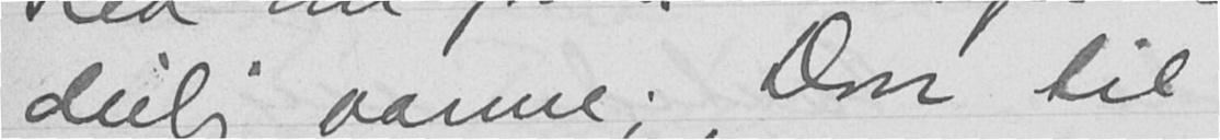deilig varme; Don til
